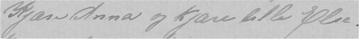Kjære Anna og kjære lille Else.
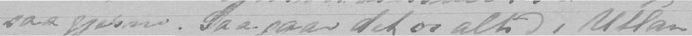saa gjerne. Saa gaar det os altid i Utlan-
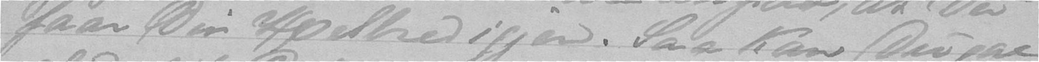faar Din Helbred igjen. Saa kan Du gaa
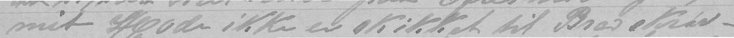mit Hode ikke er skikket til Brevskriv-
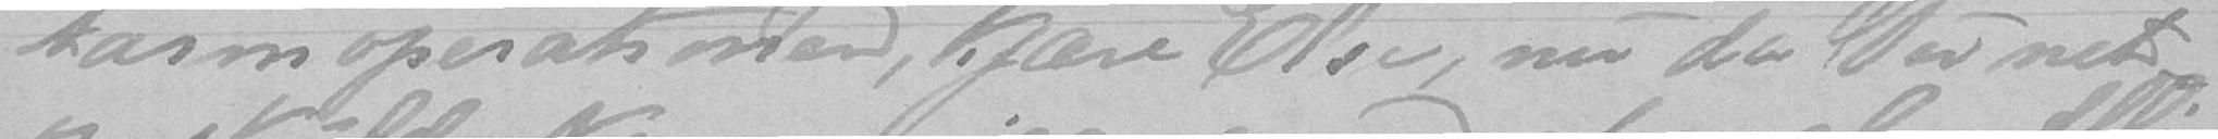tarmoperationen, kjære Else, nu da Du net-
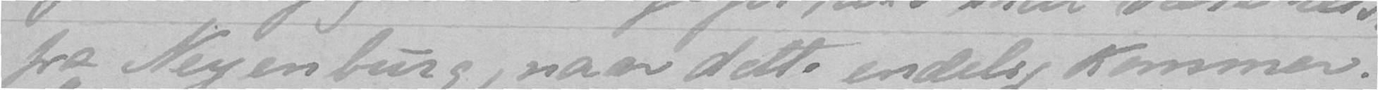fra Neyenburg, naar dette endelig kommer.
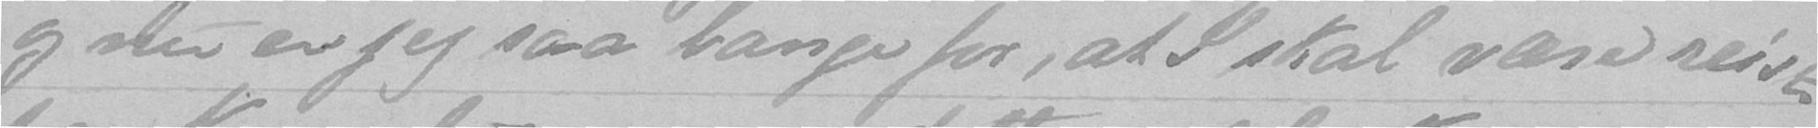og nu er jeg saa bange for, at I skal være reist
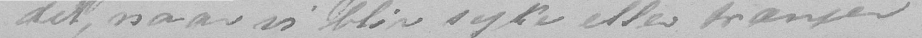det, naar vi blir syke eller trænger
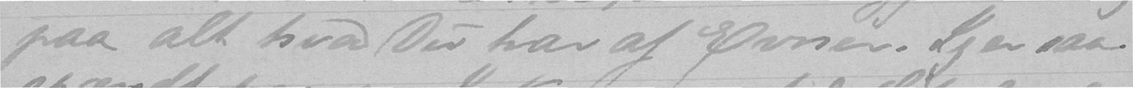paa alt hvad Du har af Evner. Jeg er saa
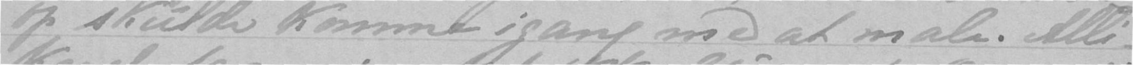op skulde komme igang med at male. Alli-
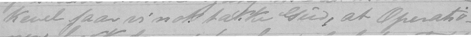kevel faar vi nok takke Gud, at Operatio-
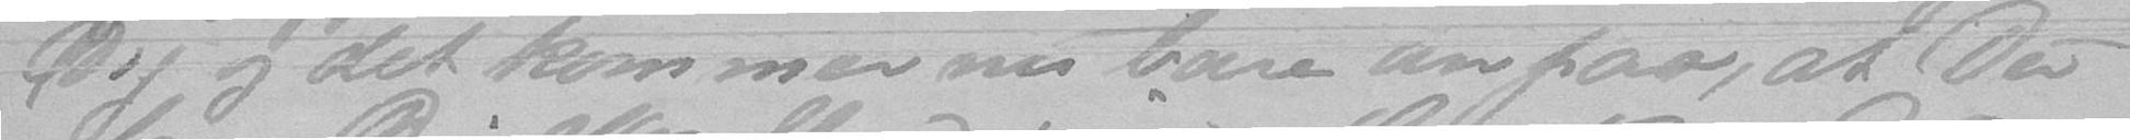Dig, og det kommer nu bare an paa, at Du
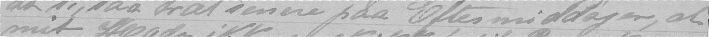at si, saa træt senere paa Eftermiddagen, at
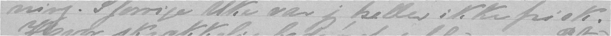ning. I forrige Uke var jeg heller ikke frisk.
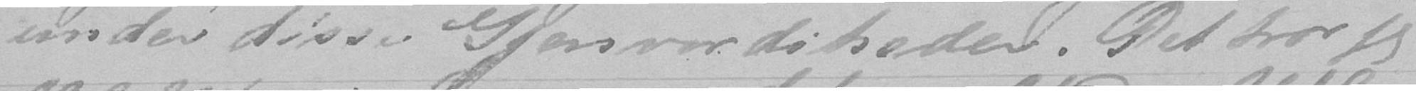under disse Gjenvordiheder. Det tror jeg
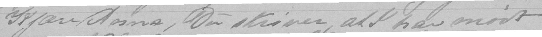Kjære Anna, Du skriver at I har mødt
